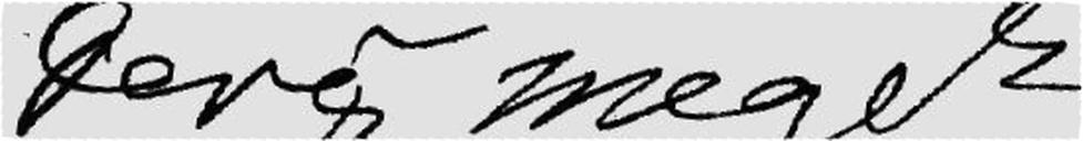Deres meget
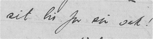sit liv for sin sak!
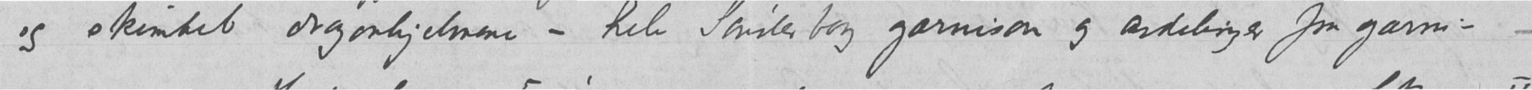og skimtet dragonhjelmene – hele Sønderborg garnison og avdelinger fra garni-
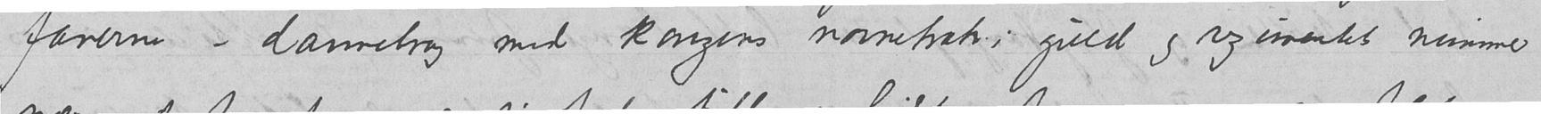fanerne – dannebrog med kongens navnetræk i guld og regimentets nummer
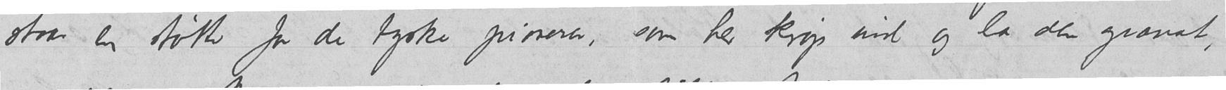staar en støtte for de tyske pionerer, som her krøp ind og la den granat,
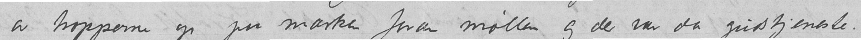av tropperne op paa marken foran møllen og der var da gudstjeneste.
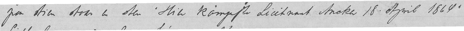paa stien staar en sten "Hier kämpfte Lieutnant Ancker 18. April 1864"
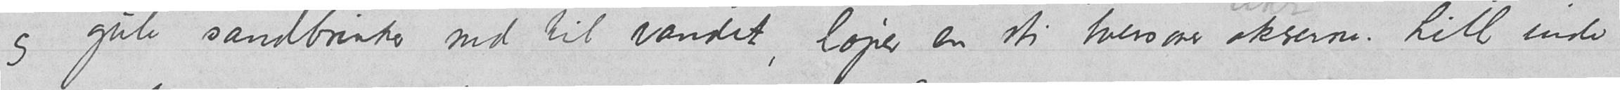og gule sandbanker ned til vandet, løper en sti tversover akrerne. Litt inde
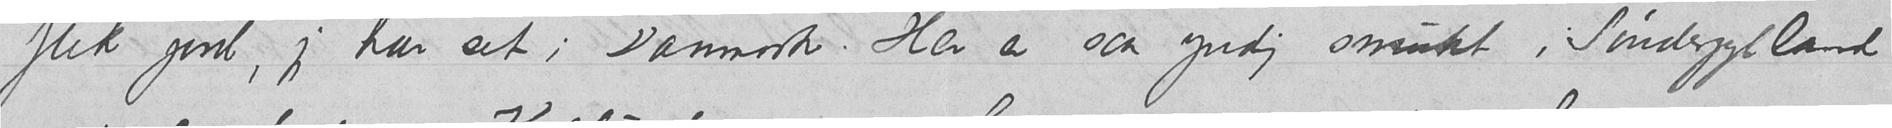flek jord, jeg har set i Danmark. Her er saa yndig, smukt i Sønderjylland
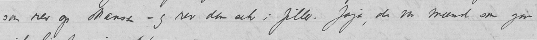som rev op skansen – og rev dem selv i filler. Jaja, de var mænd som gav
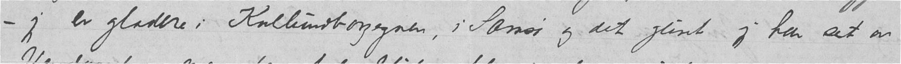– jeg er gladere i Kallundborgegnen, i Samsø og det glimt jeg har set av
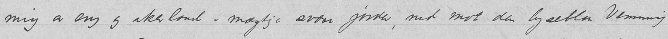ning av eng og akerland – mægtige svære jorder, ned mot den lyseblaa Vemming
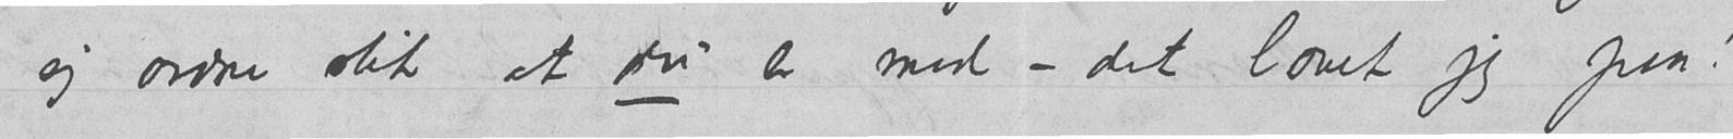sig ordne slik at du er med – det lovet jeg fruen!
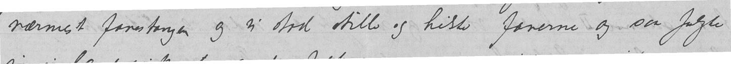nærmest fanestangen og vi stod stille og hilste fanerne og saa fulgte
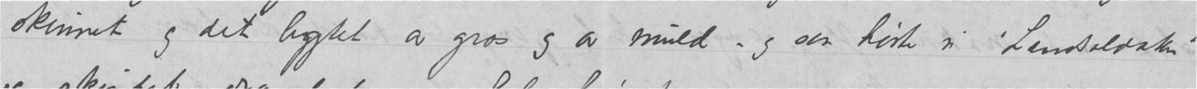skinnet og det lugtet av græs og av muld – og saa hørte vi "Landsoldaten"
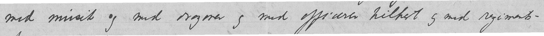med musik og med dragoner og med officerer tilhest og med regiments-
